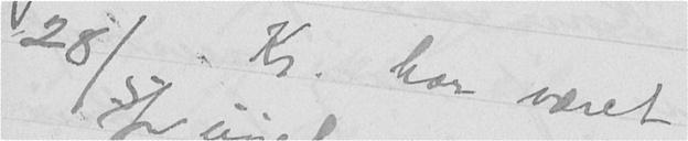28/5 Kr. har været
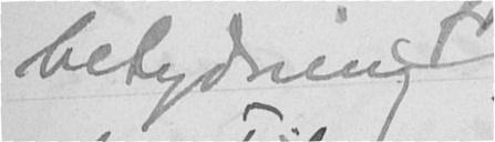betydning
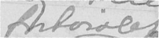fortvivlet
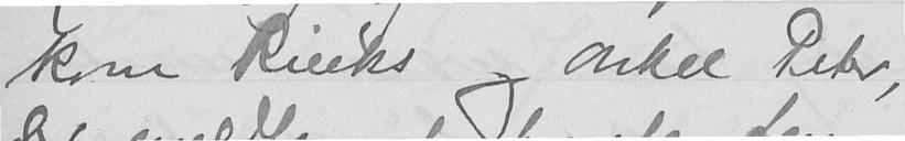kom Riecks og onkel Peter,
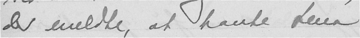der meldte, at tante Lena
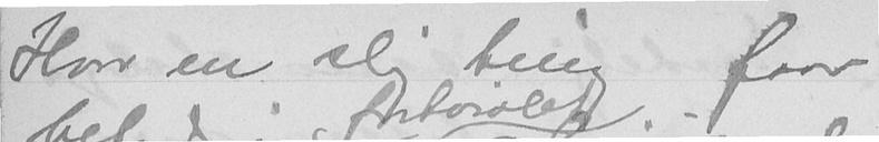Hvor en slig ting faar
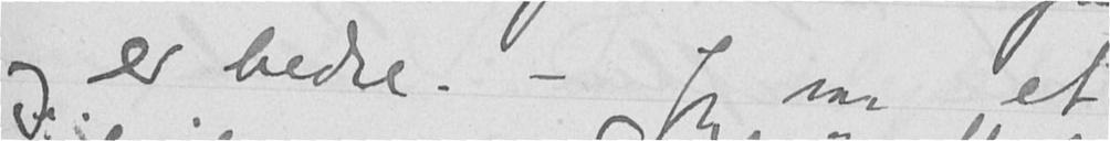og er bedre. - Jeg var et
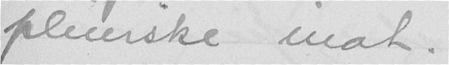pleiersken inat.
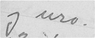og uro.
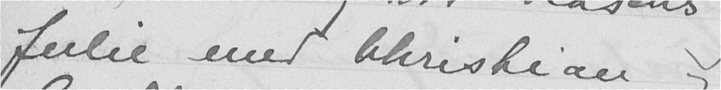Julie med Christian og
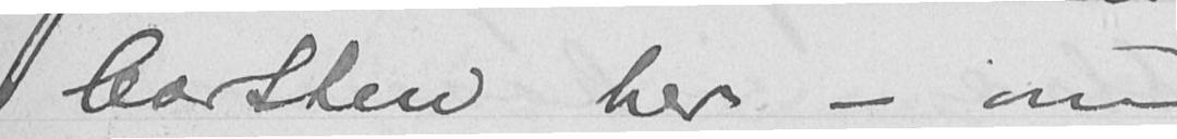Carsten her - om
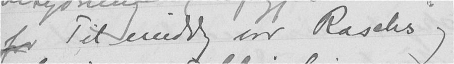for Til middag var Raschs og
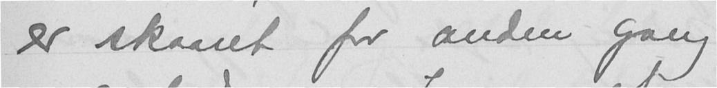er skaaret for anden gang
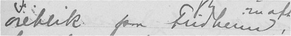øieblik paa Fridheim,
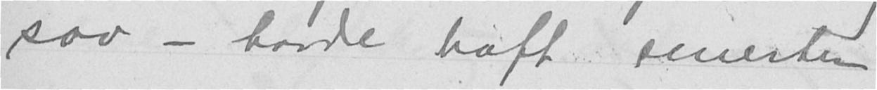sov - havde haft smerter
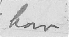hvor
